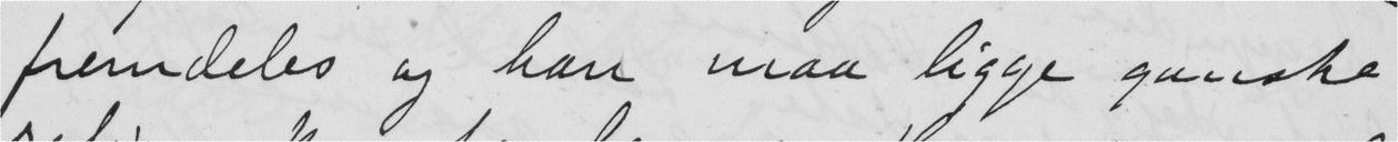fremdeles og han maa ligge ganske
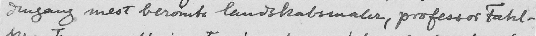dengang mest berømte landskabsmaler, professor Fahl-
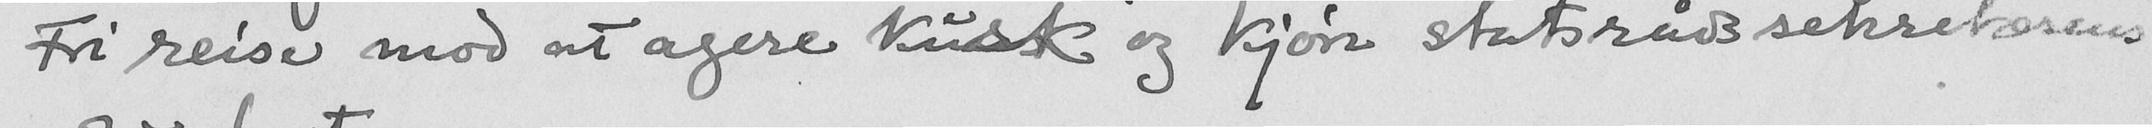Fri reise mod at agere kusk og kjøre statsrådssekretærens
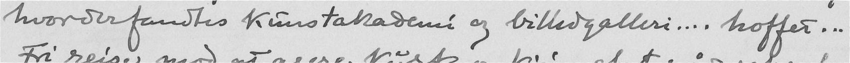hvorderfandtes kunstakademi og billedgalleri... hoffet...
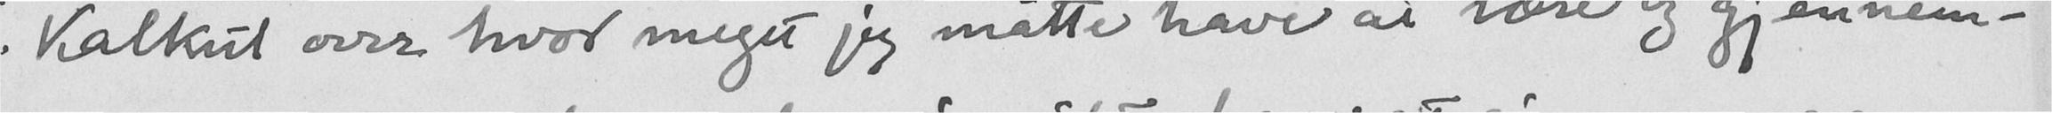...Kalkul over hvor meget jeg måtte have at lære og gjennem-
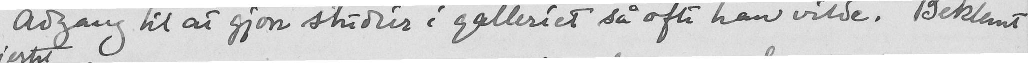Adgang til at gjøre studier i galleriet så ofte han vilde. Beklemt
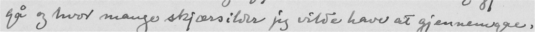gå og hvor mange skjærsilder jeg vilde have at gjennemgae,
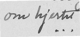om hjertet
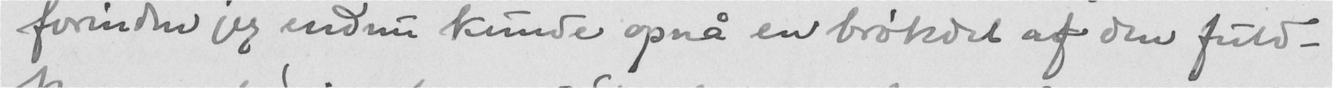forinden jeg endnu kunde opnå en brøkdel af den fuld-
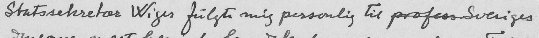Statssekretær Wiger fulgte mig personlig til profess Sveriges
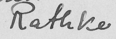Rathke
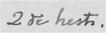2de heste.
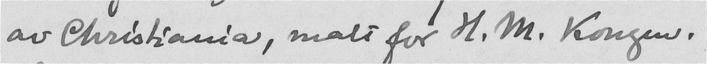av Christiania, malt for H. M. Kongen.
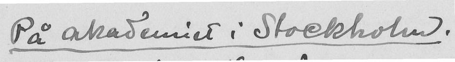På akademiet i Stockholm.
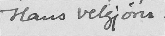Hans velgjøres
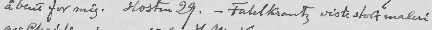åbent for mig. Hosten 29. - Fahlkrantz viste stort maleri
